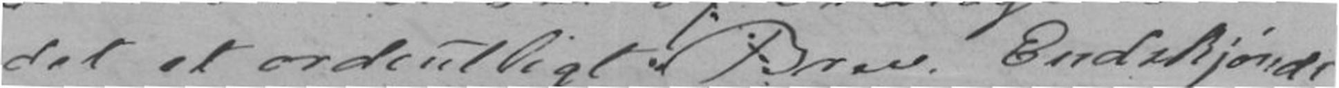det et ordentligt Brev. Endskjøndt
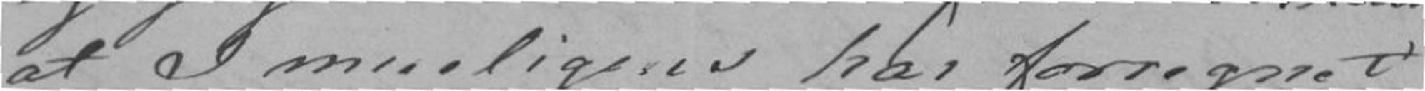at I mueligens har forregnet
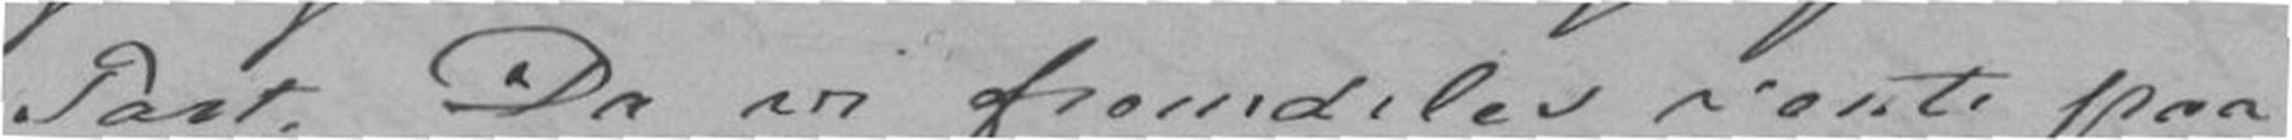Post. Da vi fremdeles vente paa
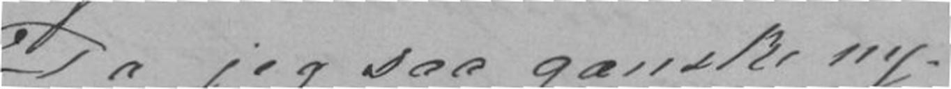Da jeg saa ganske ny-
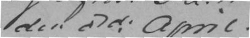den 8de April.
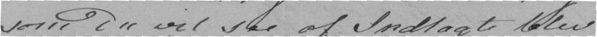som Du vil see af Indlagt blev
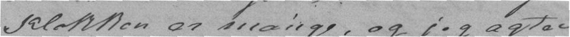Klokken er mange, og jeg agter
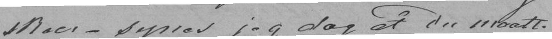skeer - synes jeg dog at Du maatte
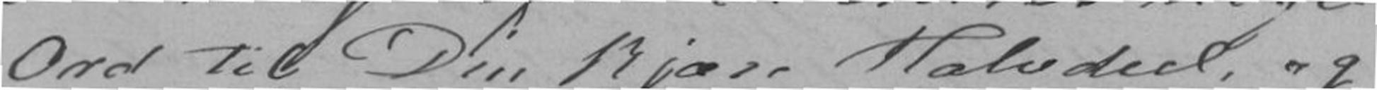Ord til Din kjære Halvdeel, og
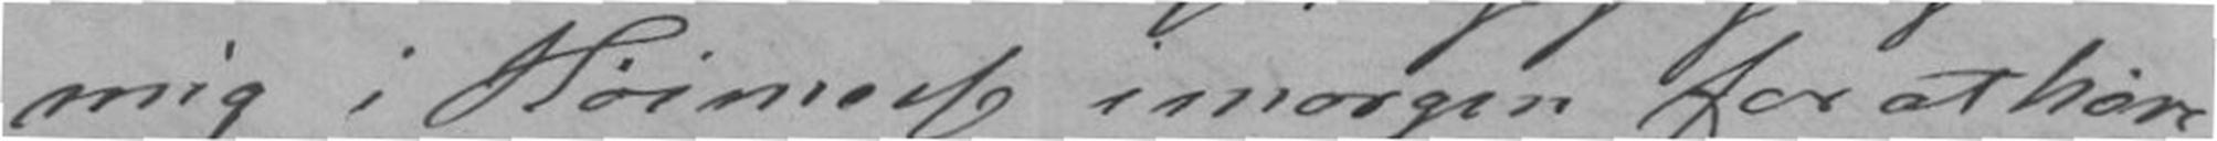mig i Høimesse imorgen for at høre
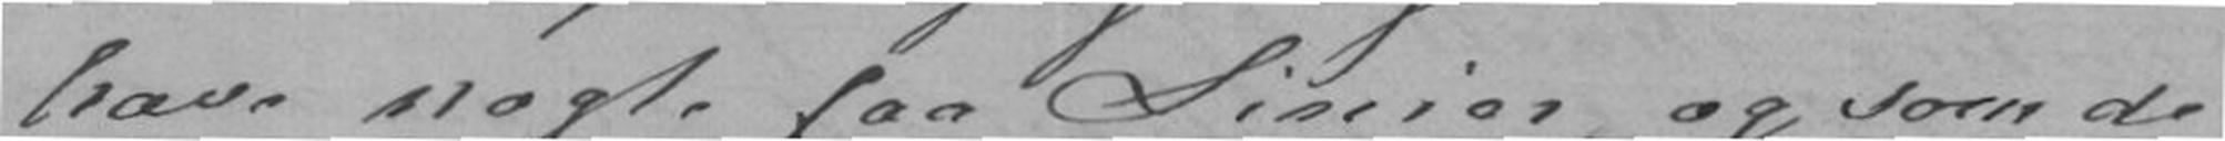have nogle faa Linier, og som de
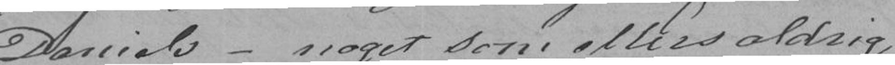Daniels - noget som ellers aldrig
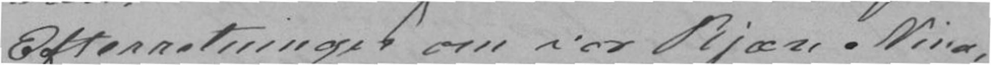Efterretninger om vor kjære Nina,
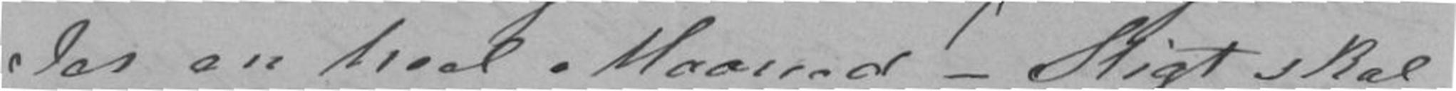Jer en heel Maaned - Sligt skal
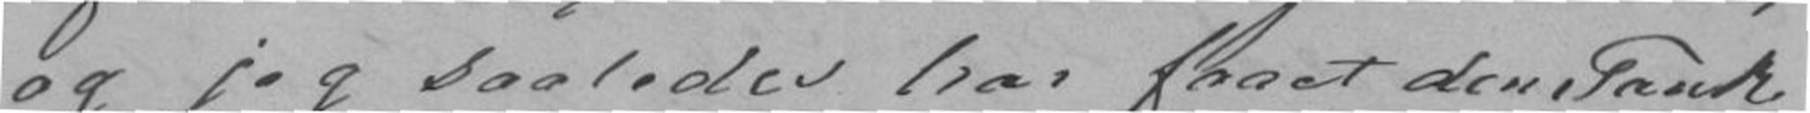og jeg saaledes har faaet den Tanke
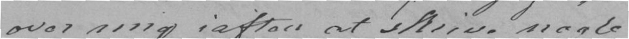over mig iaften at skrive nogle
